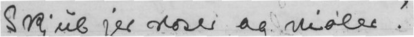Skjul jer roser og violer!
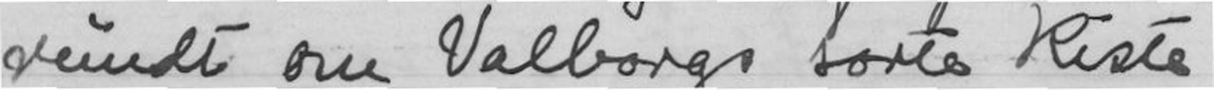rundt om Valborgs sorte kiste -
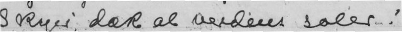Skyer, dør al verdens soler!
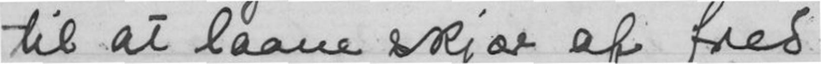til at laane skjær af fred
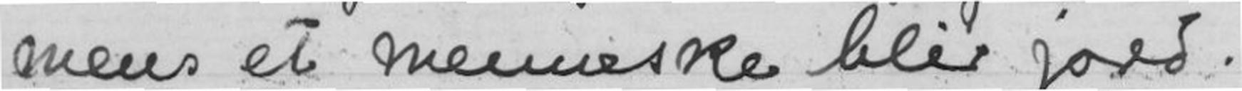mens et menneske blir jord.
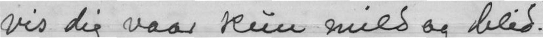vis dig vaar kun mild og blid!
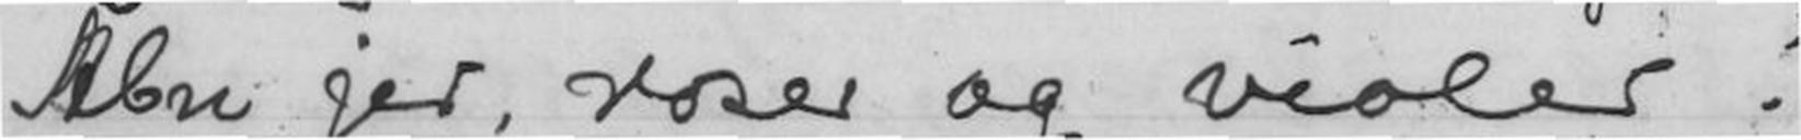Åbn jer, roser og violer!
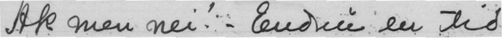Ak men nei! Endnu en tid
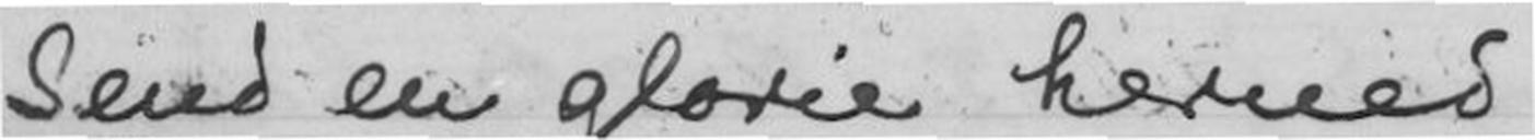Send en glorie herned
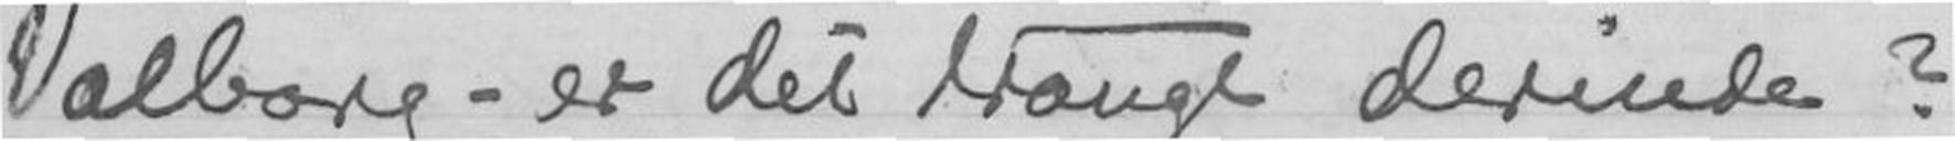Valborg - er det trangt derinde?
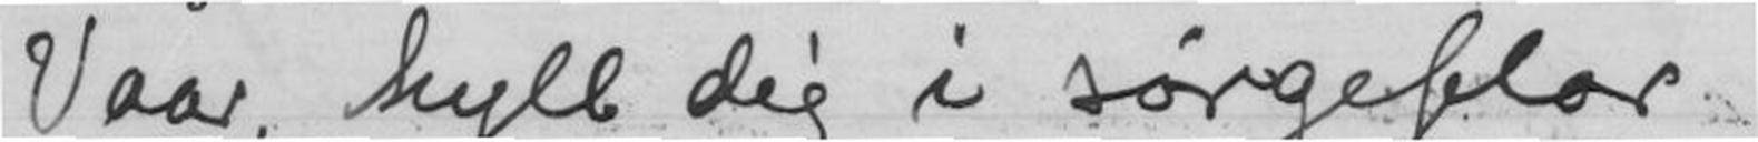Vaar, hyll dig i sørgeflor
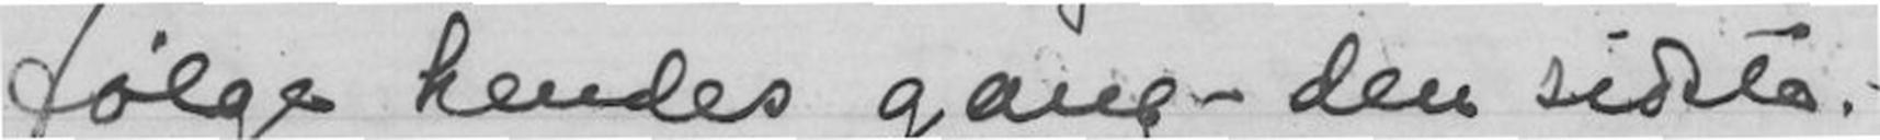følge hendes gang - den sidste. -
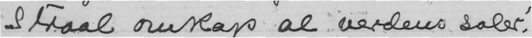Straal omkap al verdens soler!
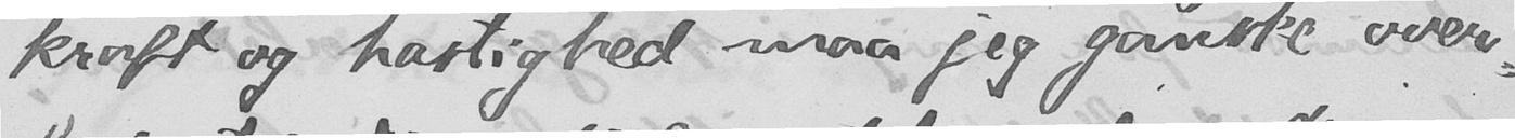kraft og hastighed maa jeg ganske over-
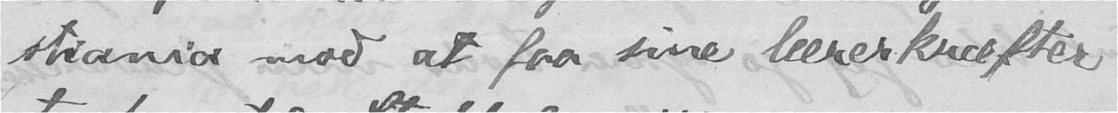stiania mod at faa sine lærerkræfter
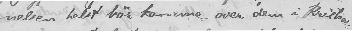nelsen helst bør komme over dem i Kristia-
